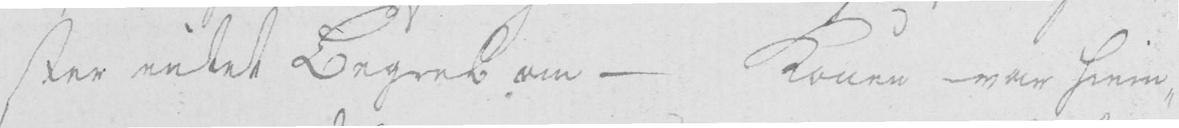ster intet Begreb om - Konen var hiem-
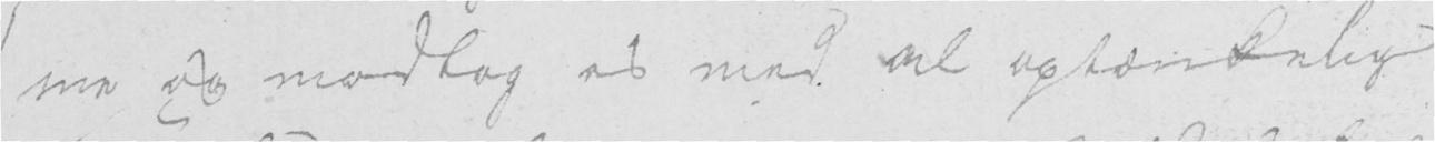me og modtog os med al optænkelig
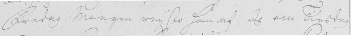Fredag Morgen reyste han af og om Tirsdag
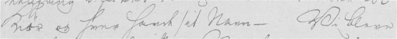Kiør og hver havde sit Navn - Vi bleve
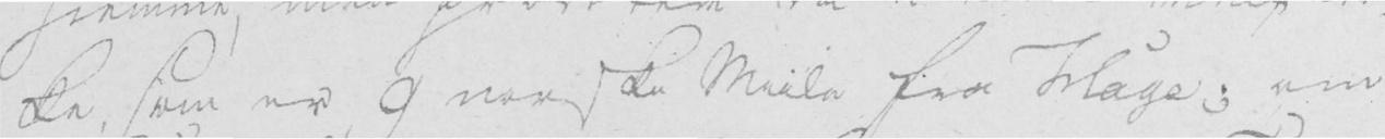ke som er 9 norske Miile fra Mage; om
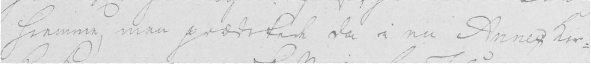hiemme, men prædikede da i en Annen Kir-
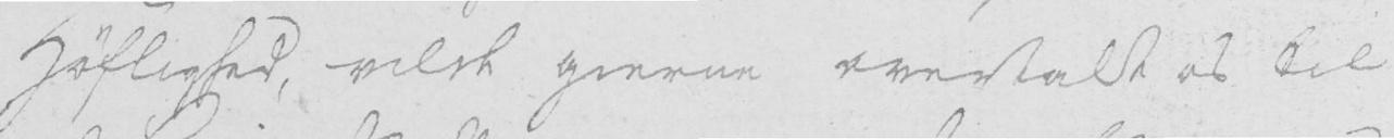Høflighed, vilde gierne overtalt os til
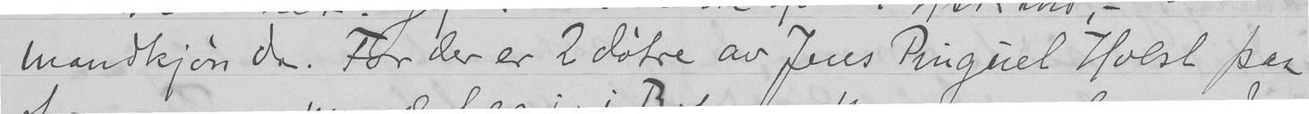mandkjønd. For der er 2 døtre av Jens Penguet Holst paa
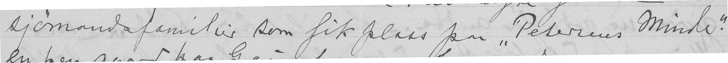sjømandsfamiler som fik plass paa "Petersens Minde".
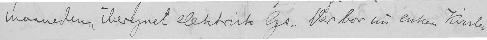maaneden, iberegnet elektrisk Gas. Der bor nu enken Kirsten
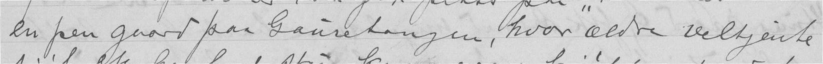en pen gaard paa Gausetangen, hvor ældre velkjente
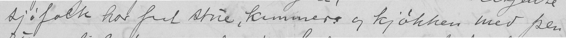sjøfolk har faat stue, kammers og kjøkken med pen
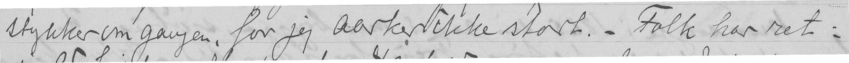stykker om gangen, for jeg aarker ikke stort. - Folk har ret -
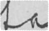ta
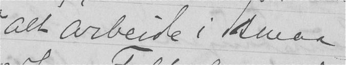alt arbeide i smaa
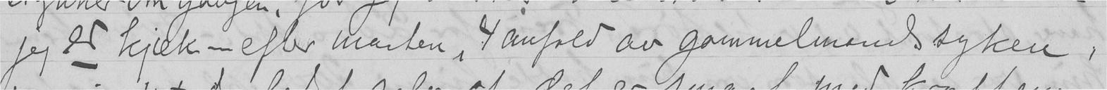jeg er kjæk - efter maaten, 4 anfald av gammelmandssyken,
